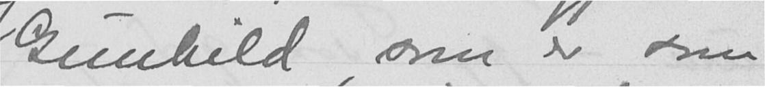Gunhild, som er som
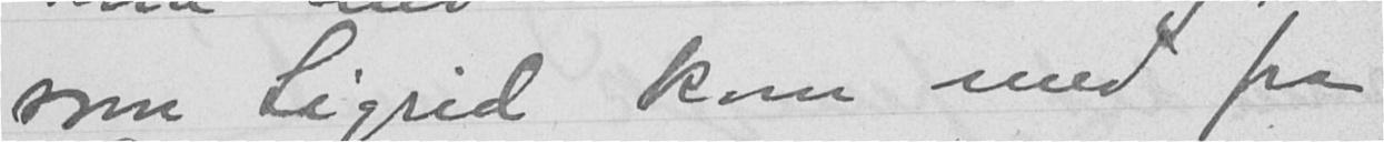som Sigrid kom med fra
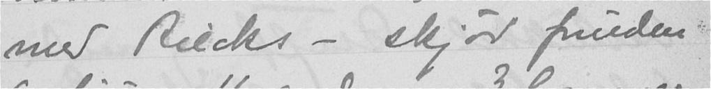med Riecks - skjød foruden
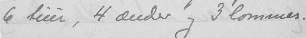6 tiur, 4 ænder og 3 Iommer.
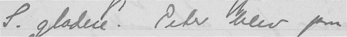S. gladere. Peter blev paa
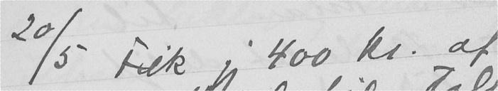20/5 Fikk jeg 400 kr. af
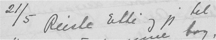21/5 Reiste Etti og jeg til
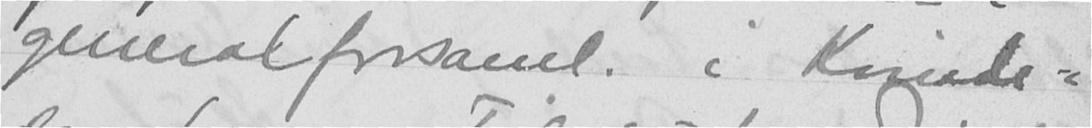generalforsaml. i Kvinde-
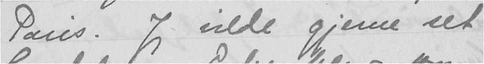Paris. Jeg vilde gjerne set
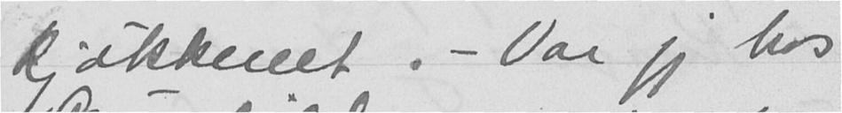kjøkkenet. - Var jeg hos
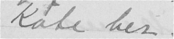Kate her.
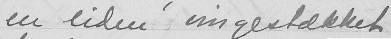en liden vingestækket
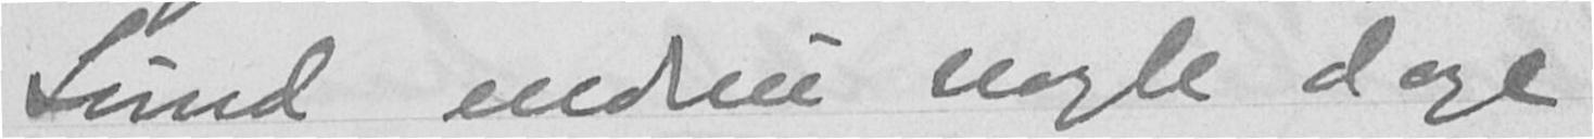Sund endnu nogle dage
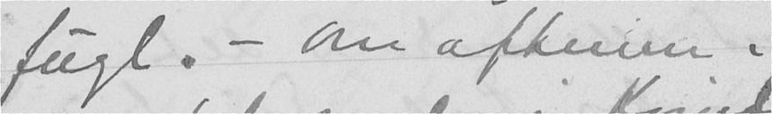fugl. - Om aftenen i
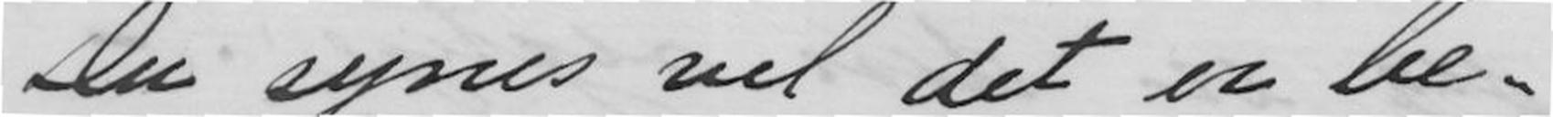Du synes vel det er be-
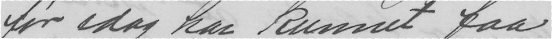før idag har kunnet faa
